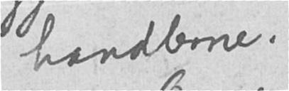handlerne.
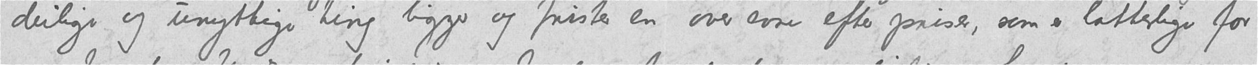deilige og unyttige ting ligger og frister en over ævne efter priser, som er latterlige for
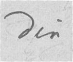Din
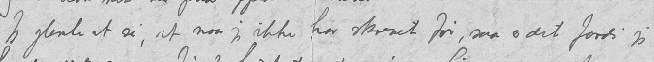Jeg glemte at si, at naar jeg ikke har skrevet før, saa er det fordi jeg
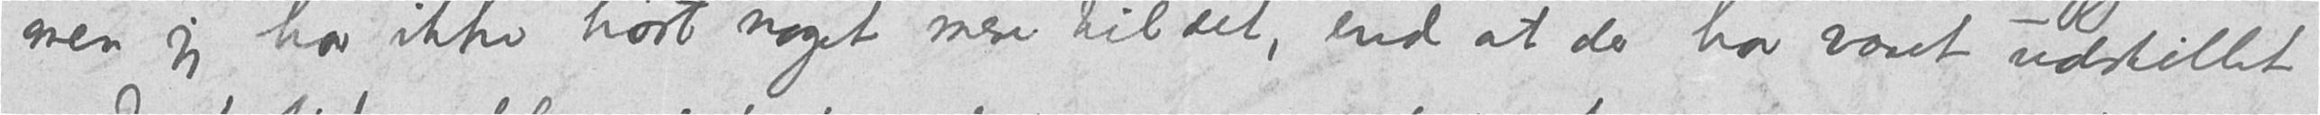men jeg har ikke hørt noget mere til det, end at der har været udstillet
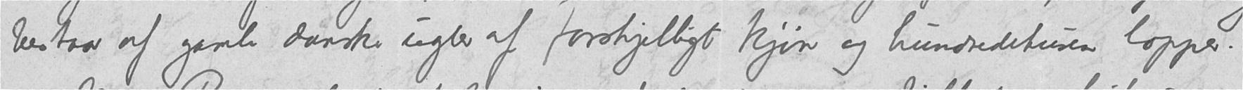bestaar af gamle danske ugler at forskjelligt kjøn og hundredetusen lopper.
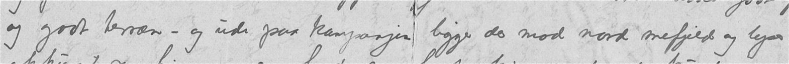og godt terræn – og ude paa kampanjen  ligger der mod nord snefjeld og løyper
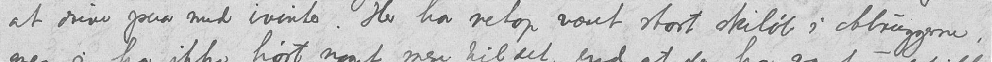at drive paa med ivinter. Her har netop været stort skiløb i Abruggerne,
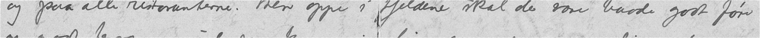og paa alle ristoranterne. Men oppe i fjeldene skal der være baade godt føre
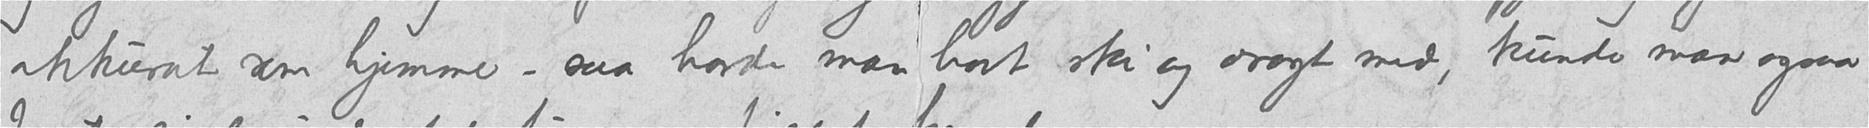akkurat som hjemme – saa havde man havt ski og dragt med, kunde man ogsaa
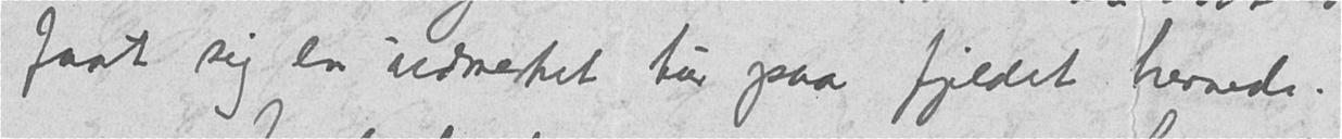faat sig en udmerket tur paa fjeldet hernede.
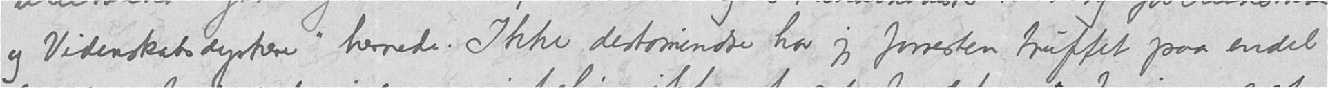og Videnskabsdyrkere" hernede. Ikke destomindre har jeg forresten truffet paa endel
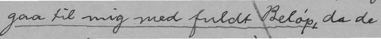gaa til mig med fuldt Beløp, da de
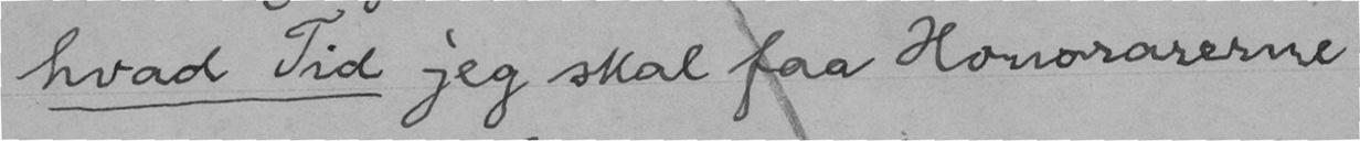hvad Tid jeg skal faa Honorarerne
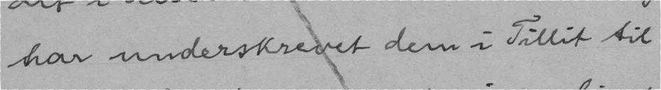har underskrevet dem i Tillit til
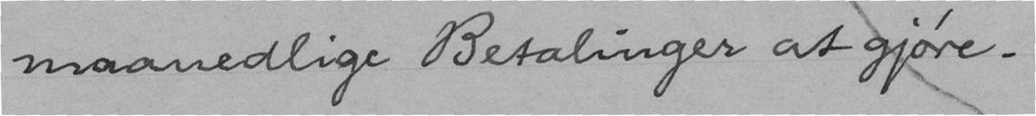maanedlige Betalinger at gjøre.
